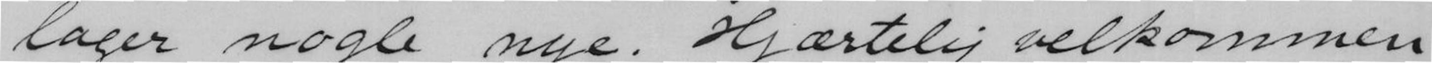lager nogle nye. Hjærtelig velkommen
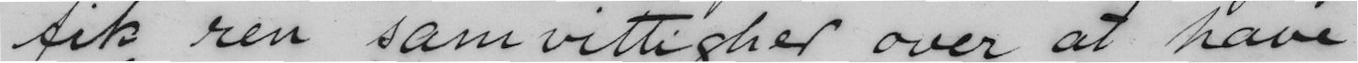fik ren samvittighed over at have
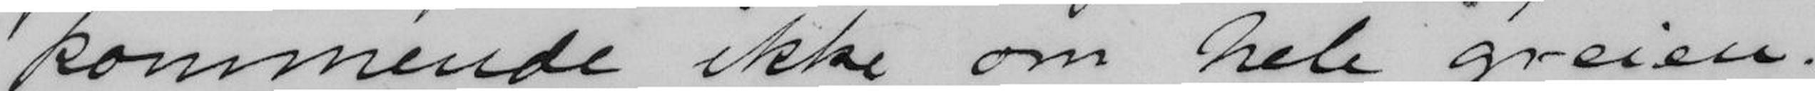kommende ikke om hele greien.
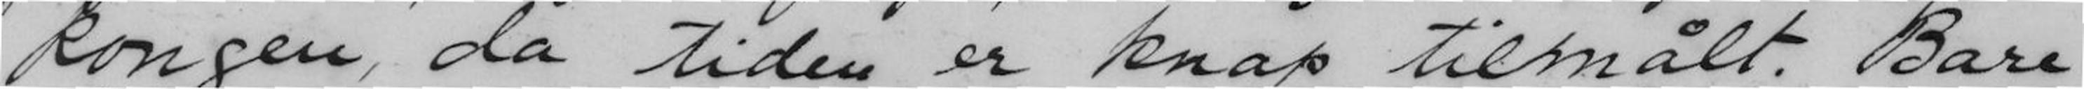kongen, da tiden er knap tilmålt. Bare
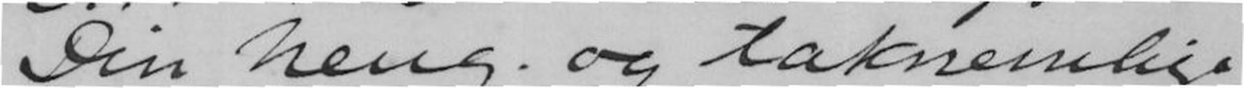Din heng. og taknemlige
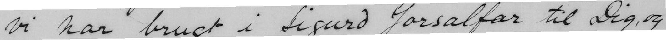vi har brugt i Sigurd Jorsalfar til Dig, og
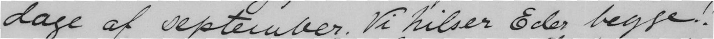dage af september. Vi hilser Eder begge!!
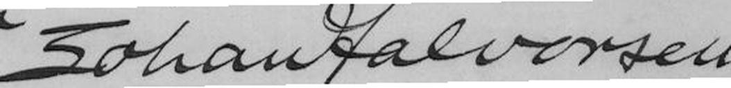Johan Halvorsen
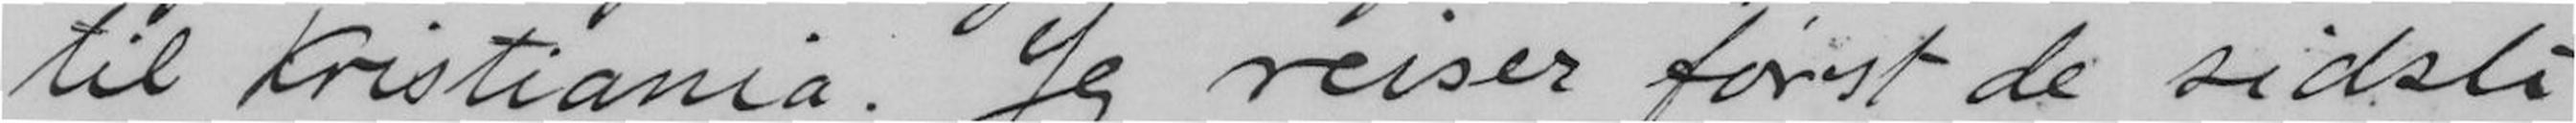til Kristiania. Jeg reiser først de sidste
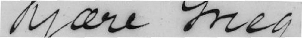Kjære Grieg!
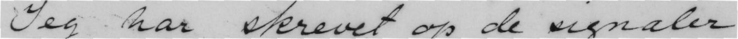Jeg har skrevet op de signaler
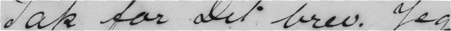Tak for Dit brev. Jeg
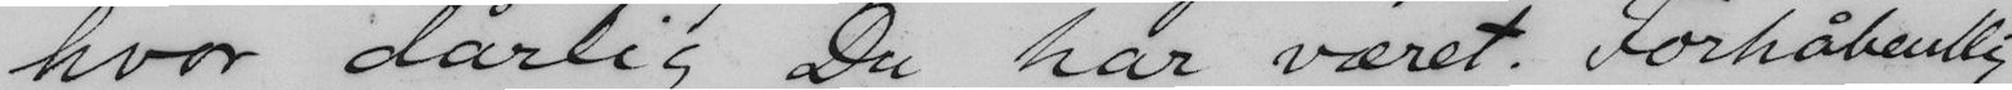hvor dårlig Du har været. Forhåbentlig
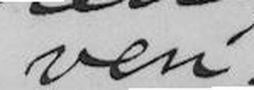ven
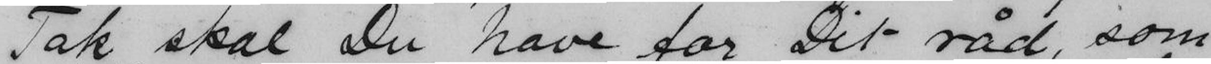Tak skal Du have for Dit råd, som
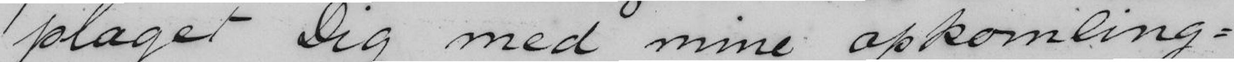plaget Dig med mine opkomling-
